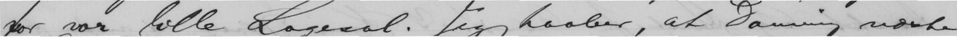for vor lille Logesal. Jeg haaber, at Danning næste
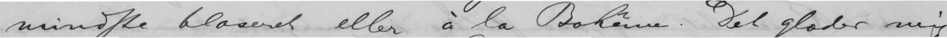mindste blaseret eller à la Bohême. Det glæder mig
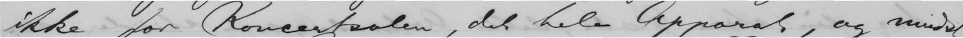ikke for Koncertsalen, det hele Apperat, og mindst
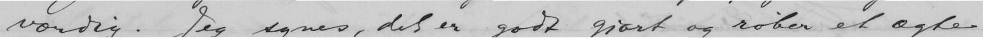værdig. Jeg synes, det er godt gjort og røber et ægte
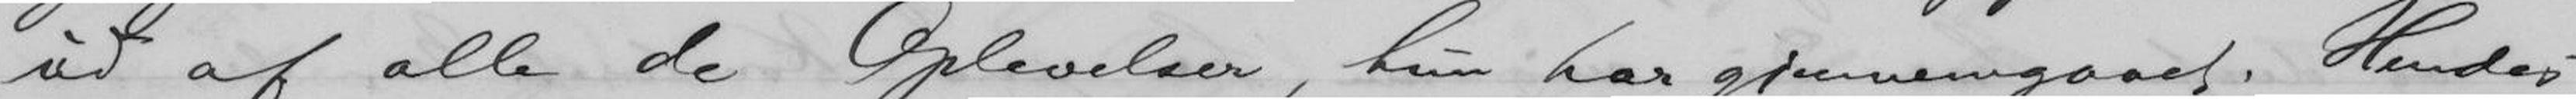ud af alle de Oplevelser, hun har gjennemgaaet. Hendes
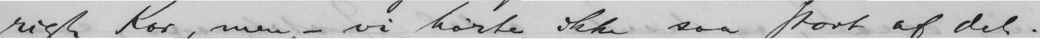rigt Kor, men - vi hørte ikke saa stort af det.
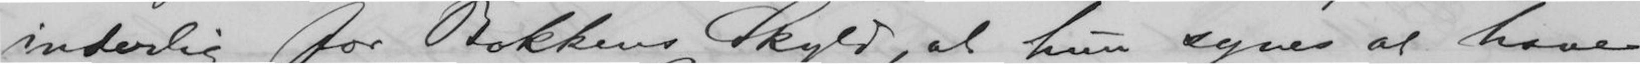inderlig for Bokkens Skyld, at hun synes at have
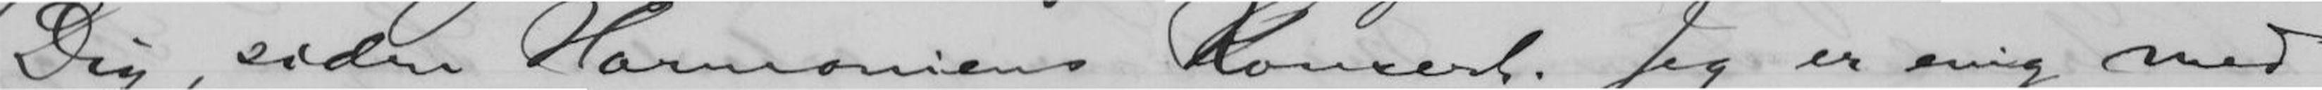Dig, siden Harmoniens Konsert. Jeg er enig med
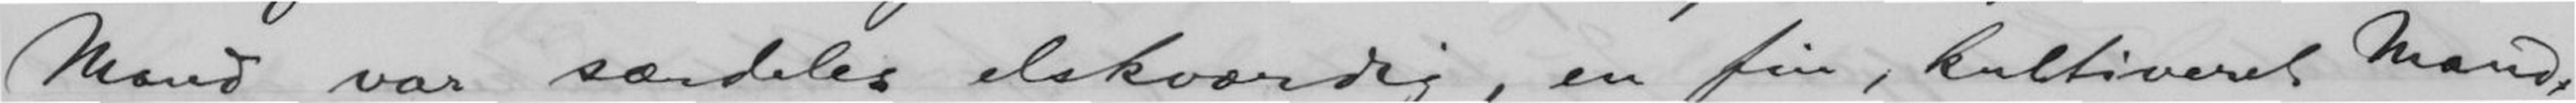Mand var særdeles elskværdig, en fin, kultiveret Mand,
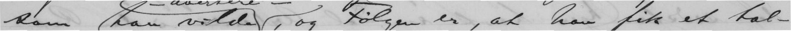som han vilde, og Følgen er, at han fik et tal-
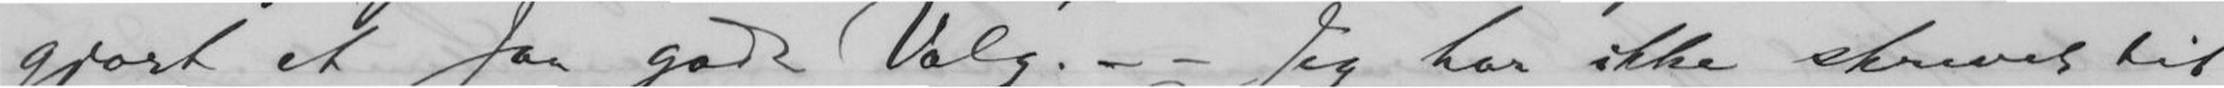gjort et saa godt Valg. - - Jeg har ikke skrevet til
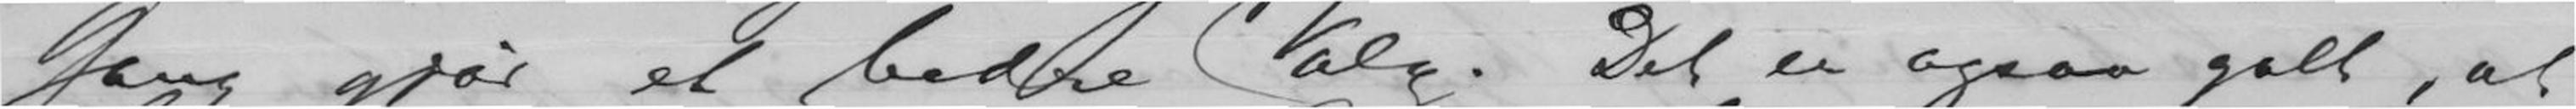Gang gjør et bedre Valg. Det er ogsaa galt, at
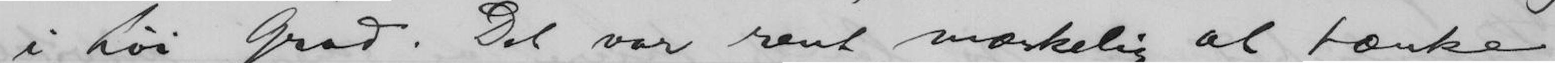i høi Grad. det var rent mærkelig at tænke
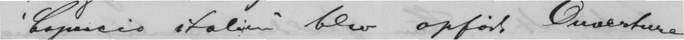"Capricio italien" blev opført Ouverture
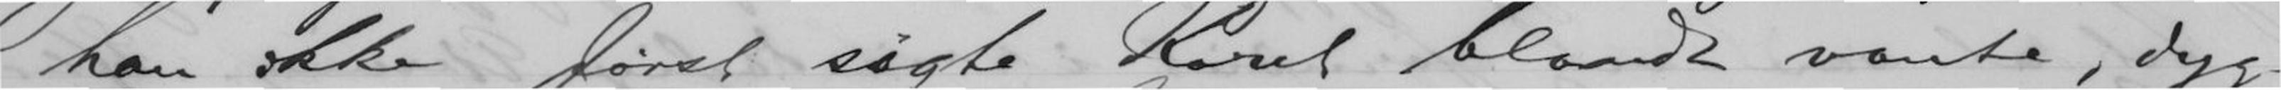han ikke først søgte Koret blandt vante, dyg-
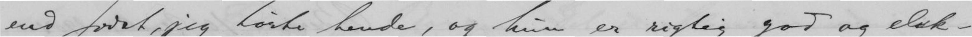end sidst, jeg hørte hende, og hun er rigtig god og elsk-
